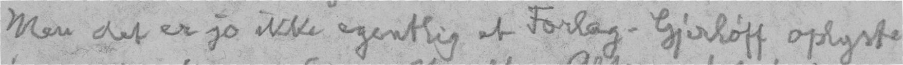Men det er jo ikke egentlig et Forlag. Gjerløff oplyste
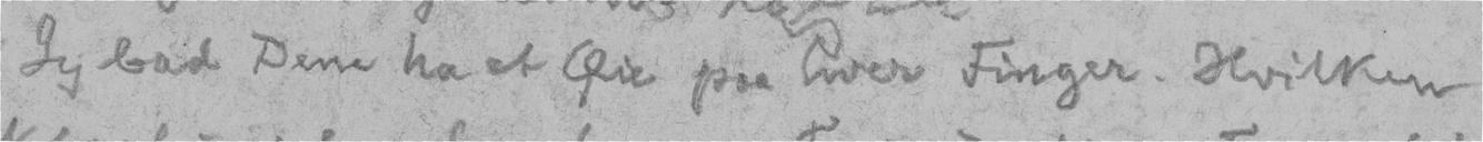Jeg bad Dem ha et Øie paa hver Finger. Hvilken
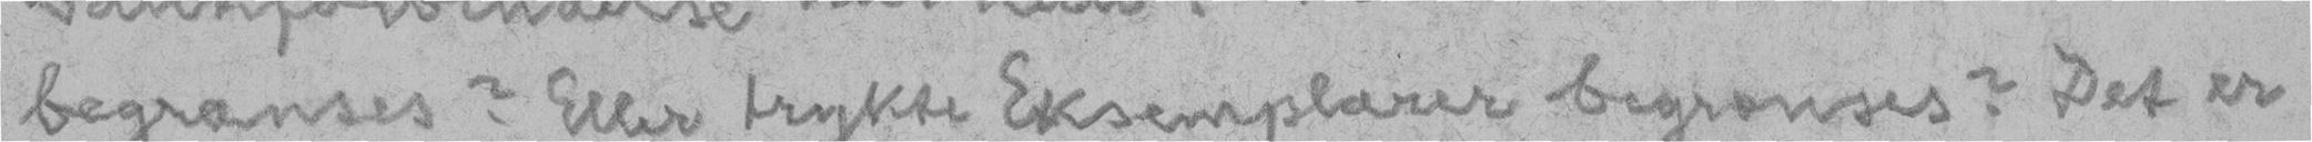begrænses? Eller trykte Eksemplarer begrænses? Det er
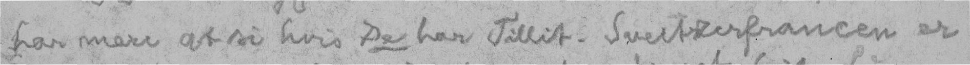har mere at si hvis De har Tillit. Sveitzerfrancen er
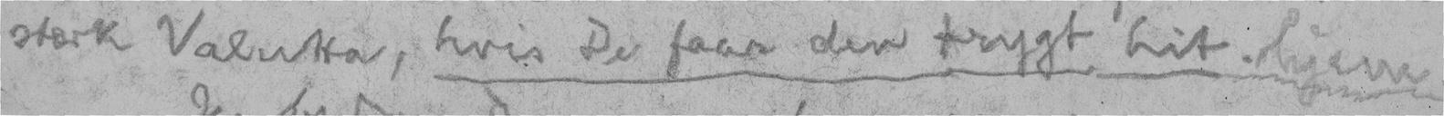stærk Valutta, hvis De faar den trygt hit. hjem
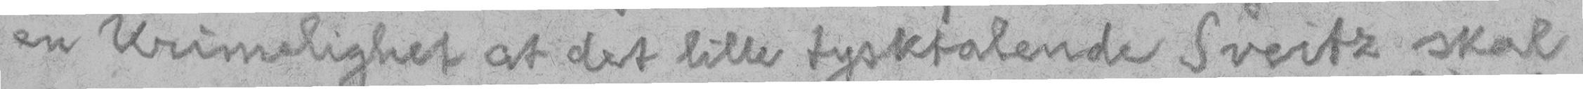en Urimelighet at det lille tysktalende Sveitz skal
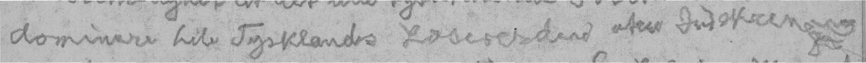dominere hele Tysklands Læseverden uten Indskrenang
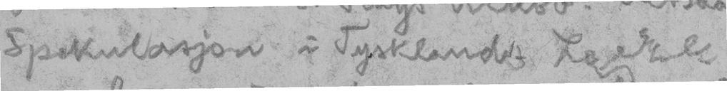Spekulasjon i Tysklands Læsere
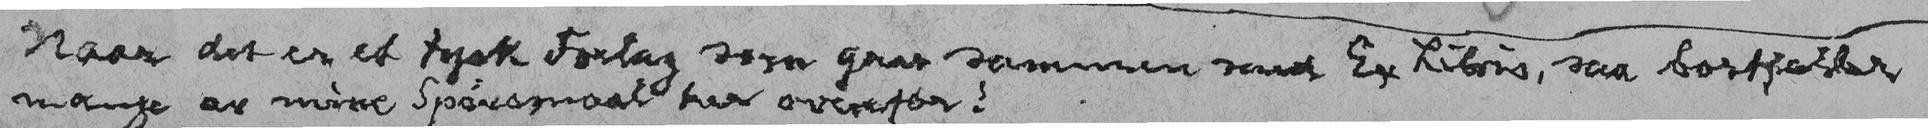Naar det er et tysk Forlag som gaar sammen med Ex Libris, saa bortfalder
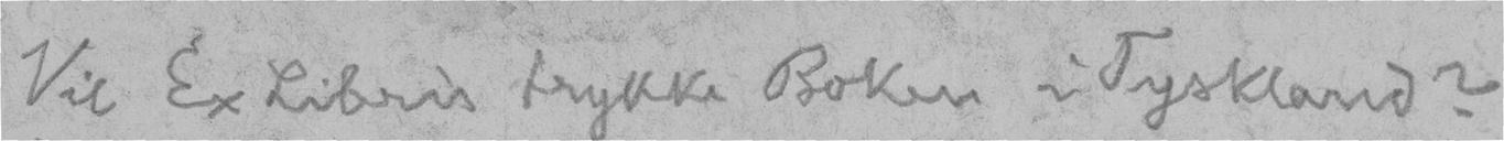Vil Ex Libris trykke Boken i Tyskland?
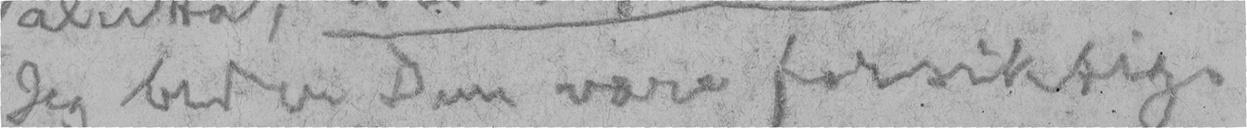Jeg beder Dem være forsiktig.
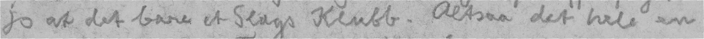jo at det bare et Slags Klubb. Altsaa det hele en
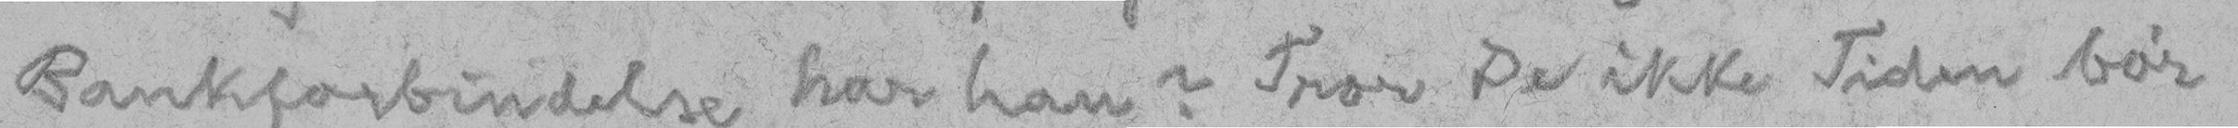Bankforbindelse har han? Tror De ikke Tiden bør
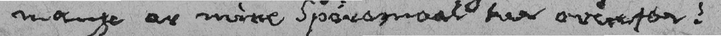mange av mine Spørsmaal her ovenfor!
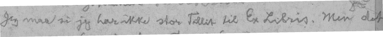Jeg maa si jeg har ikke stor Tillit til Ex Libris. Men det
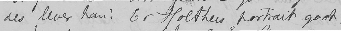des lever han? Er Holthers portrait godt,
